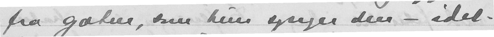fra gaten, som hun synger den - idet
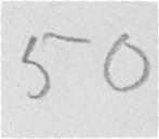50
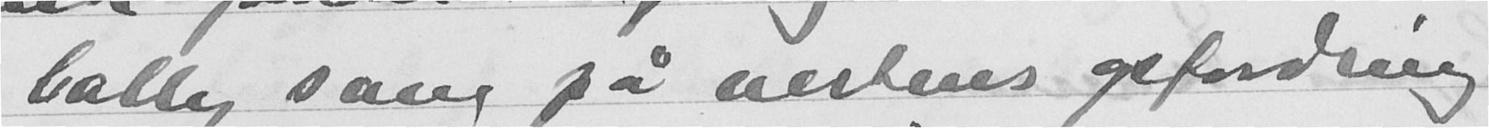Cally sang på vertens opfordring
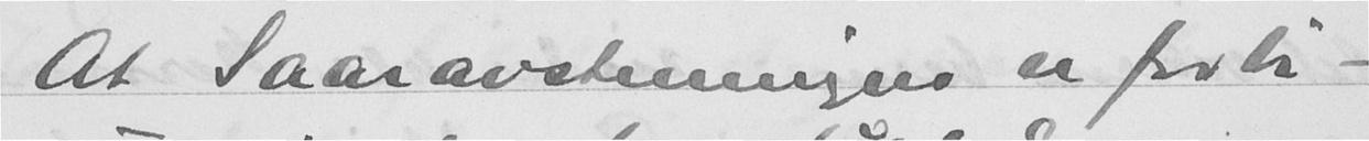At taareveningene er forbi -
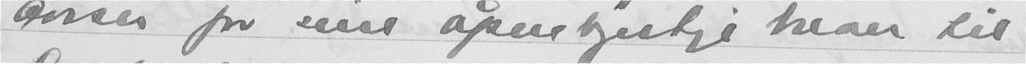aviser for sine åpenhjertige brever til
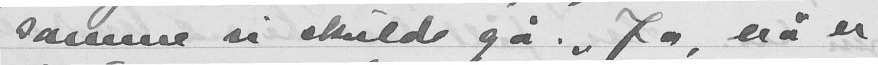samme vi skulde gå: "Ja, så er
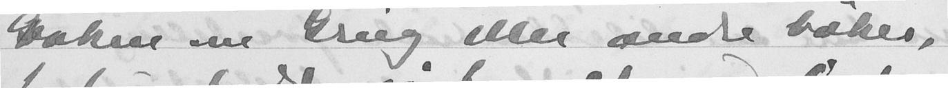boken om Grieg eller andre bøker,
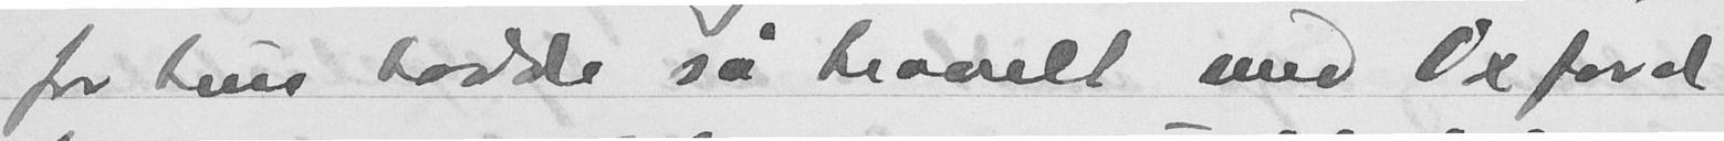for hun hadde så travelt med Oxford
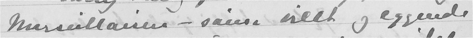Marscillanen - sam villt og eggende
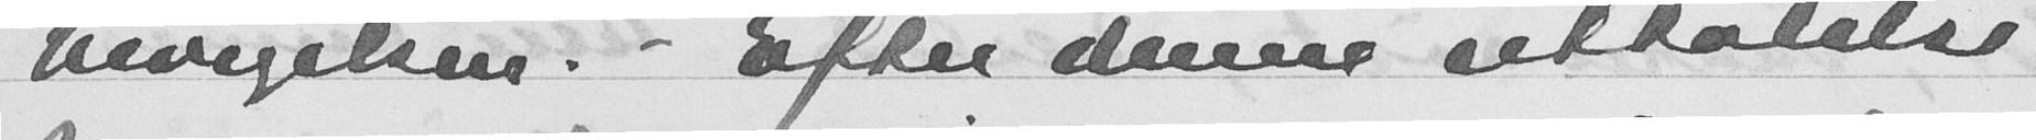bevegelsen. - Efter denne uttalelse
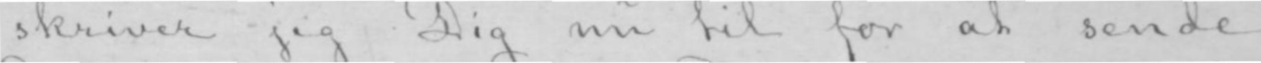skriver jeg Dig nu til for at sende
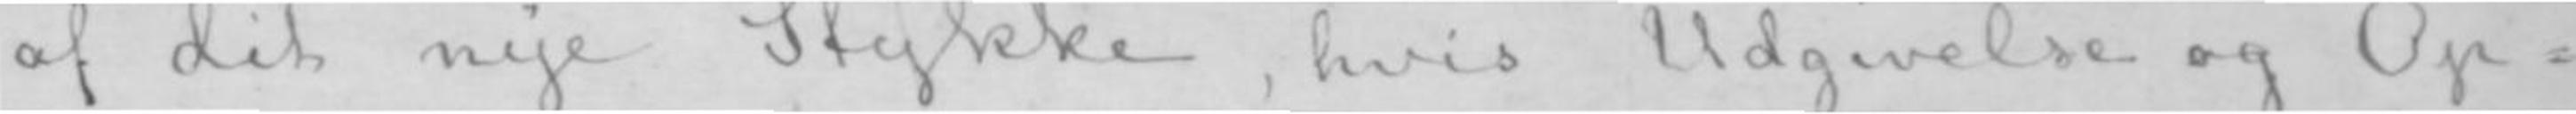af dit nye Stykke, hvis Udgivelse og Op-
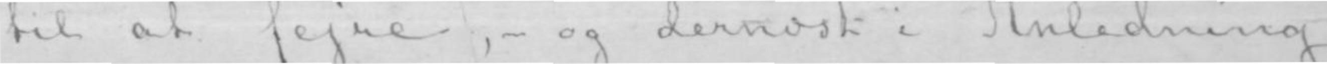til at fejre,- og dernæst i Anledning
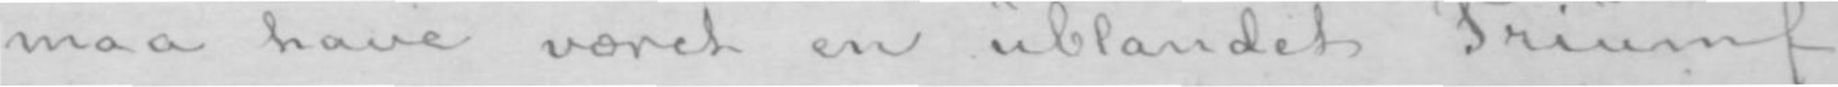maa have været en ublandet Triumf
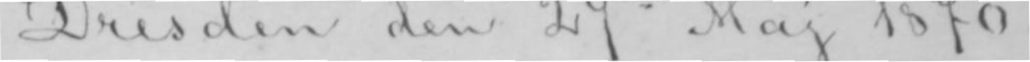Dresden den 29de Maj 1870.-
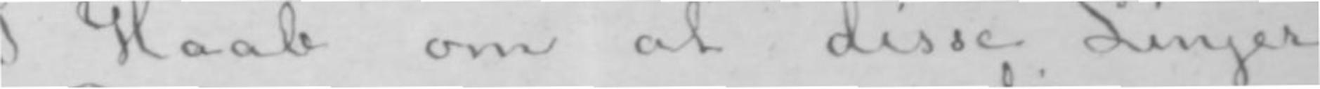I Haab om at disse Linjer
